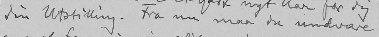din Utstilling. Fra nu maa du undvære
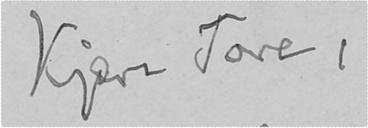Kjære Tore,
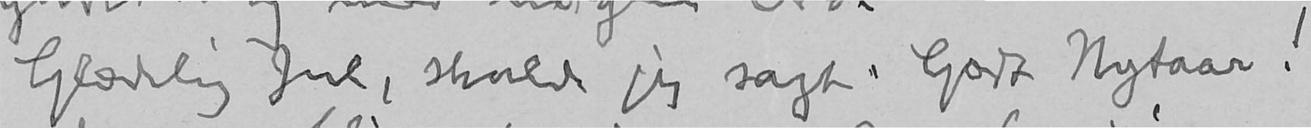Glædelig Jul, skulde jeg sagt. Godt Nytaar!
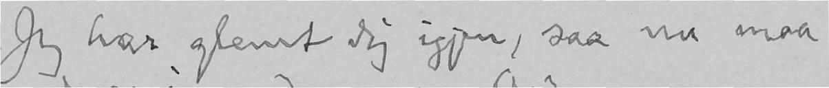Jeg har glemt dig igjen, saa nu maa
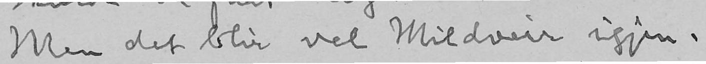Men det blir vel Mildveir igjen.
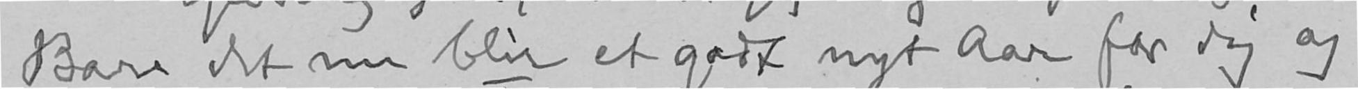Bare det nu blir et godt nyt Aar for dig og
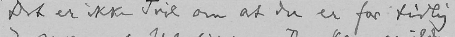Det er ikke Tvil om at du er for tidlig
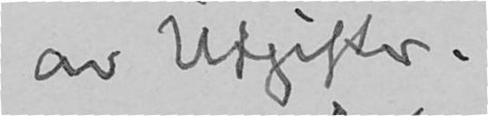av Utgifter.
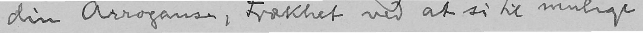din Arroganse, Frækhet ved at si til mulige
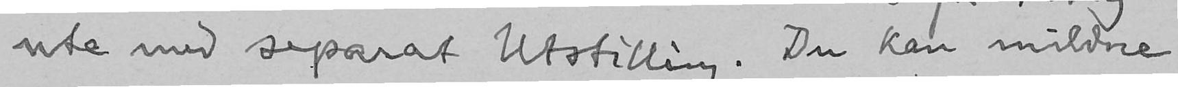ute med separat Utstilling. Du kan mildne
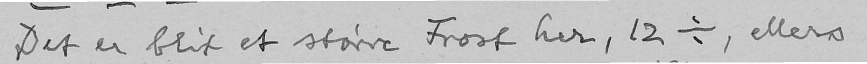Det er blit et større Frost her, 12 ÷, ellers
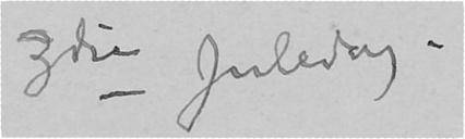3die Juledag.
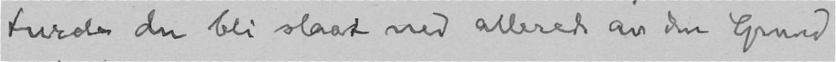turde du bli slaat ned allerede av den Grund
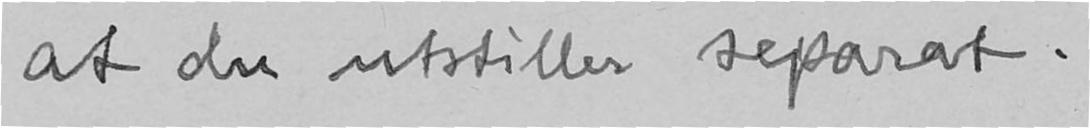at du utstiller separat.
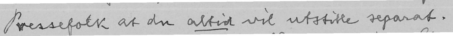Pressefolk at du altid vil utstille separat.
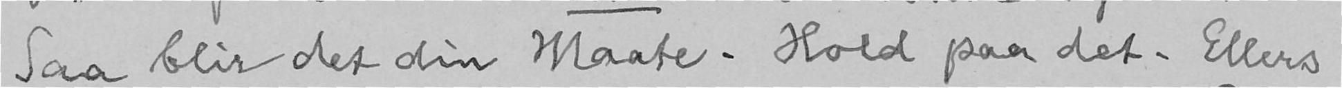Saa blir det din Maate. Hold paa det. Ellers
